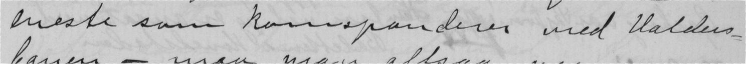eneste som korresponderer med Valders-
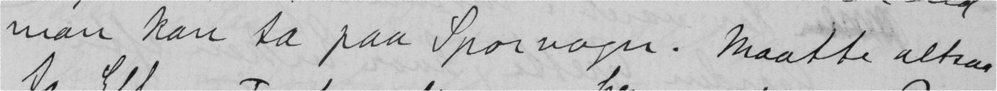man kan ta paa Sporvogn. Maatte altsaa
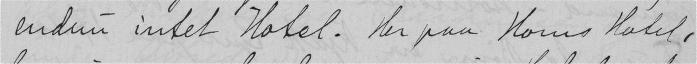endnu intet Hotel. Her paa Homs Hotel,
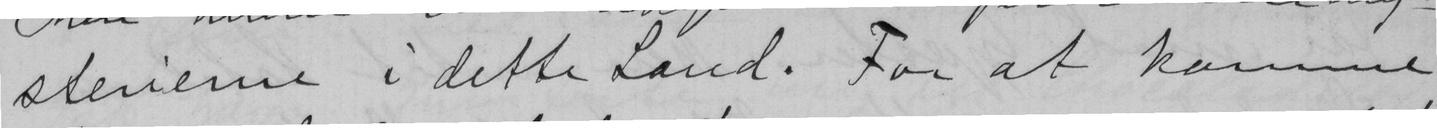sterierne i dette Land. For at komme
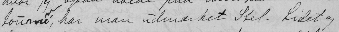tourné, har man udmærket Stel. Lidet og
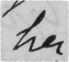her
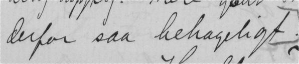derfor saa behageligt.
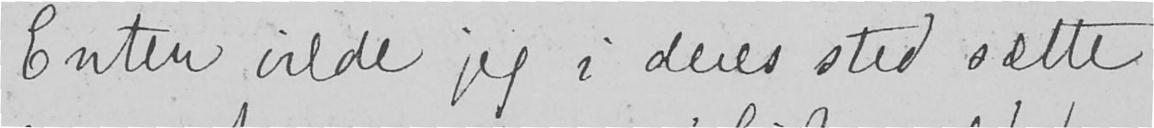Enten vilde jeg i deres sted sætte
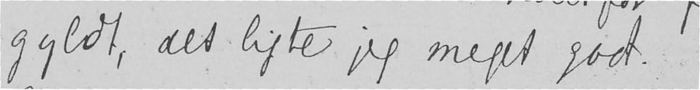gyldt, det ligte jeg meget godt.
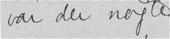var der nogle
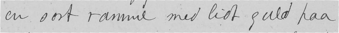en sort ramme med lidt guld paa
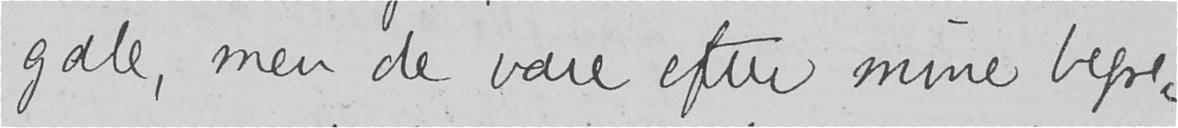gale, men de vare efter mine begre-
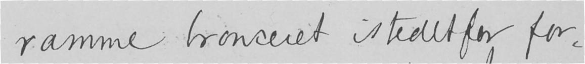ramme bronceret istedetfor for-
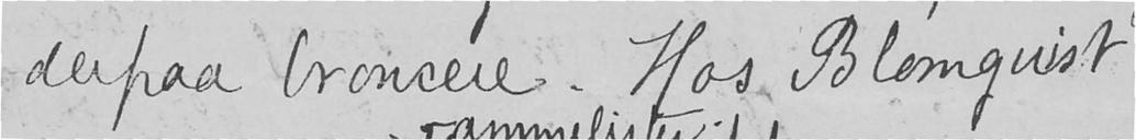derpaa broncere. Hos Blomquist
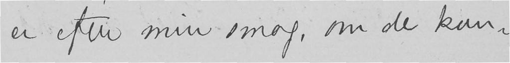er efter min smag, om de kun-
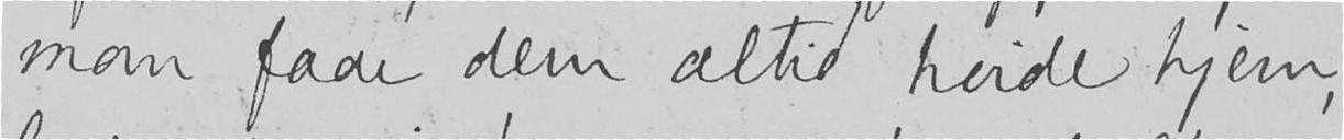man faar dem altid hvide hjem,
